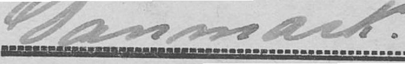Danmark.
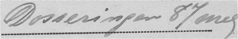Dosseringen 87 meg
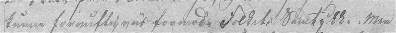kunne fornuftigviis formode Folkets Samtykke. Men
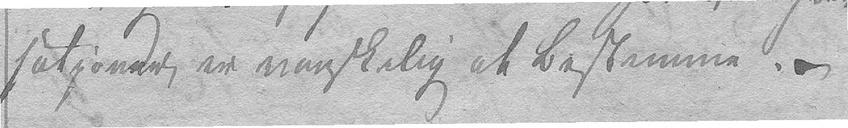sationer er vanskelig at bestemme. –
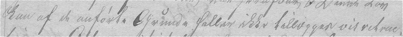kan af de anførte Grunde heller ikke tillægges vis retroac-
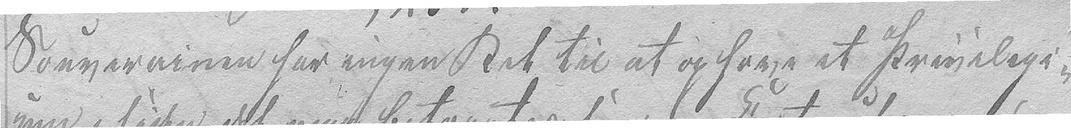Souverainen har ingen Ret til at ophæve et Privilegi-
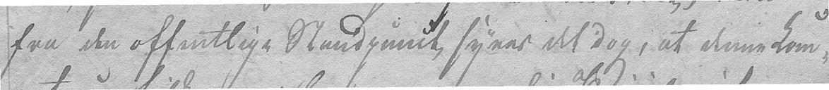fra den offentlige Standpunct synes det dog, at denne Com-
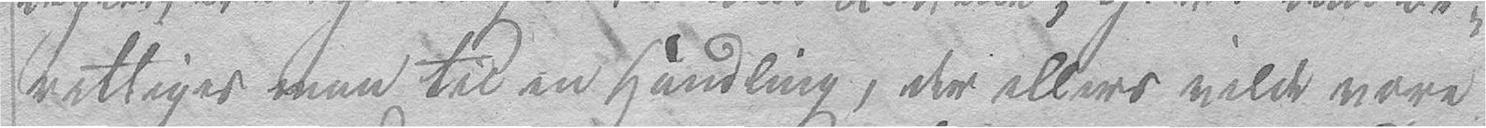rettiges man til en Handling, der ellers vilde være
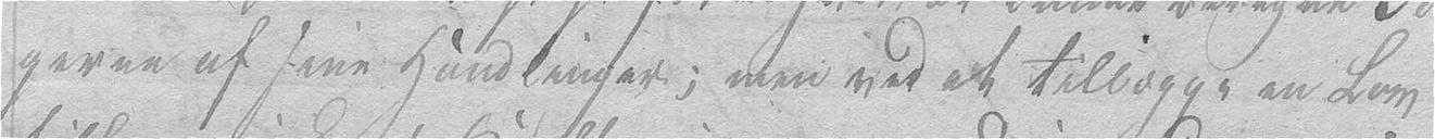gerne af sine Handlenger, men ved at tillægge en Lov
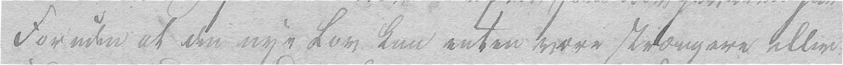Foruden at den nye Lov kan enten være strængere eller
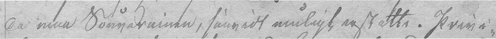da maa Souverainen, saavidt muligt erstatte. Privi-
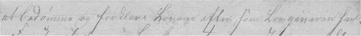at bedømme og forklare Lovene efter som Lovgiveren har.
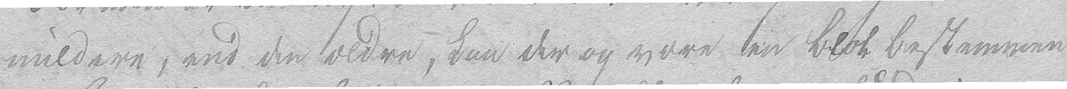mildere end de ældre, kan der og være en blot bestemmen-
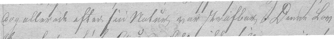dog allerede efter sin Natur var strafbar. Denne Lov
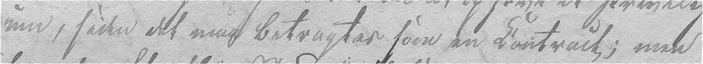um, siden det maa betragtes som en Contract; men
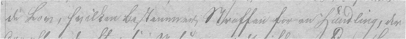de Lov, hvilken bestemmer Straffen for en Handling, der
